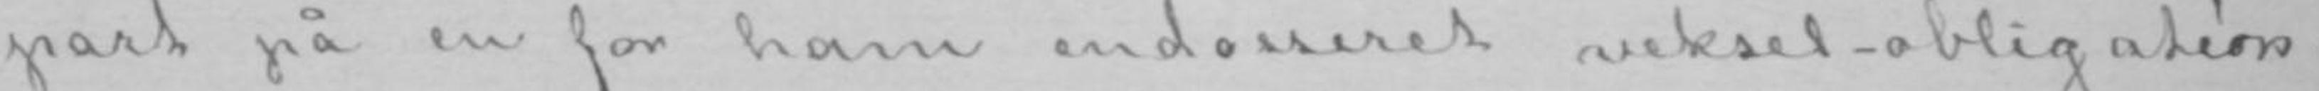part på en for ham endosseret veksel-obligation.
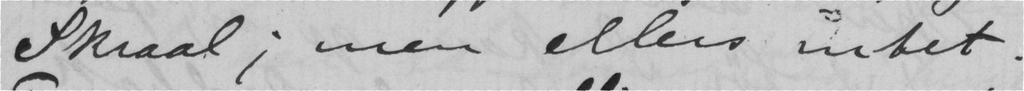Skraal; men ellers intet.
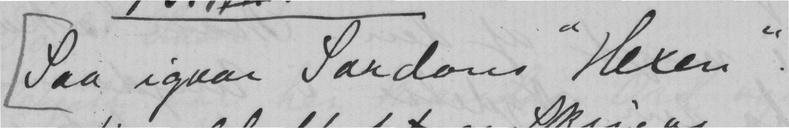Saa igaar Sardons "Hexen"
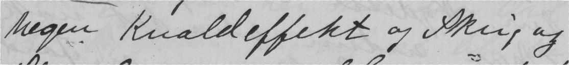Megen Knaldeffekt og Skrig og
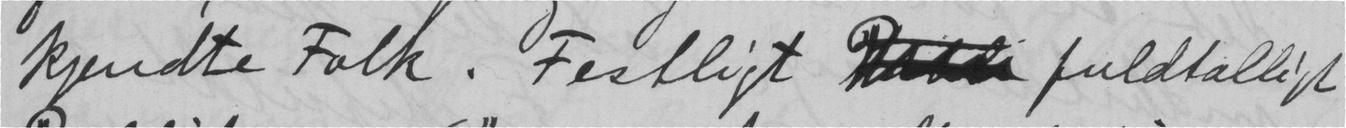kjendte Folk. Festligt  fuldtalligt
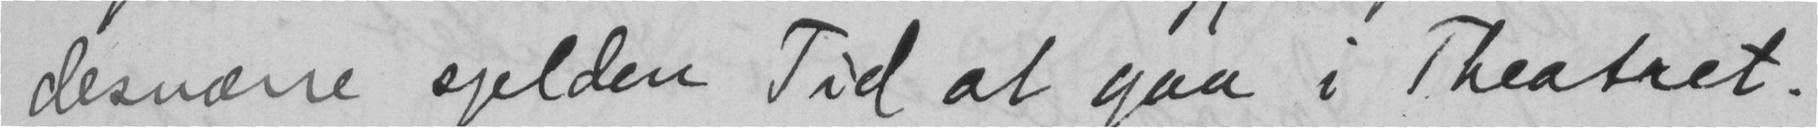desværre sjelden Tid at gaa i Theatret.
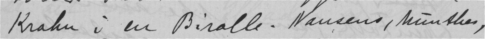Krohn i en Birolle. Nansens, Munthes,
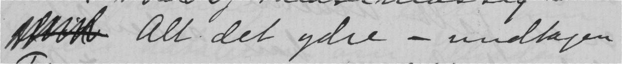Alt det ydre - undtagen
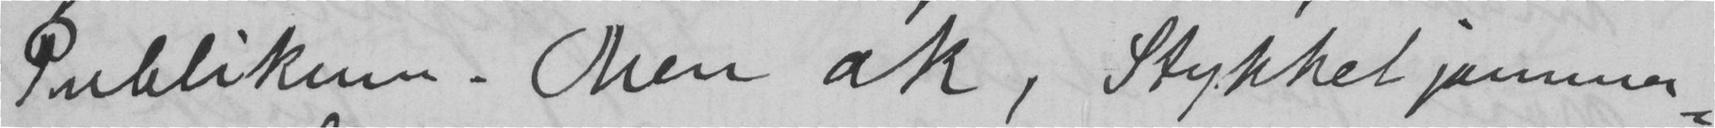Publikum. Men ak, Stykket jammer-
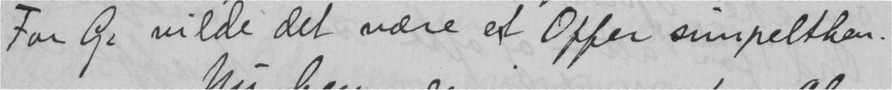For G. vilde det være et Offer simpelthen.
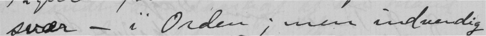svær - i Orden; men indvendig
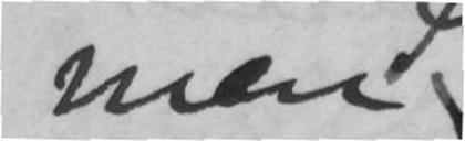men
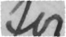for
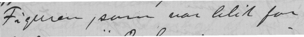Figuren, som var blit for
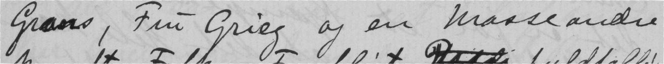Grans, Fru Grieg og en masse andre
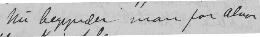Nu begynder man for alvor
